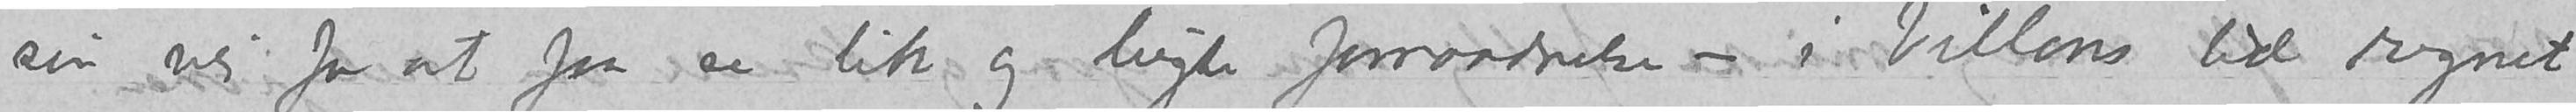sin vei for at faa se lik og lugte forraadnelse – i Villons tid regnet
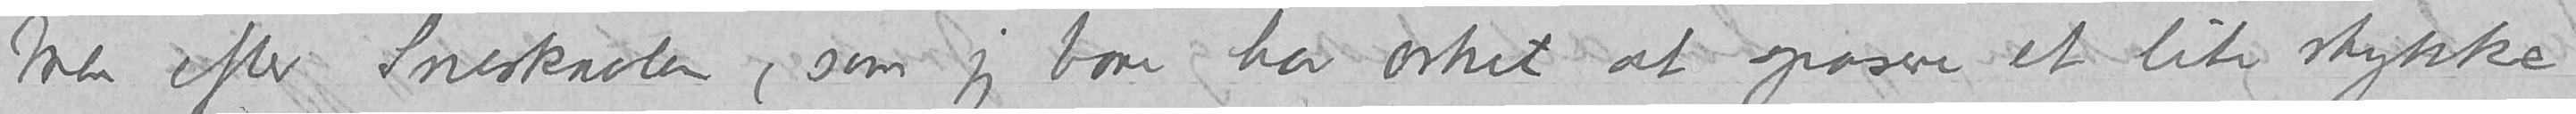Men efter Sneskavlen (som jeg bare har orket at spasere et lite stykke
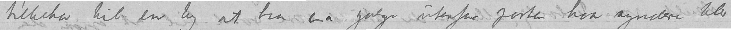tilbehør til en by at ha en galge utenfor porten hvor synderen blev
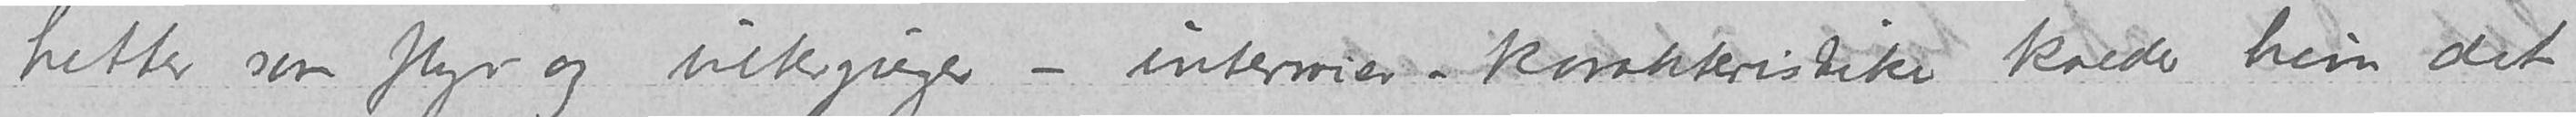hetter som flyr og intergieger - interriør-karakteristiker kalder hun det
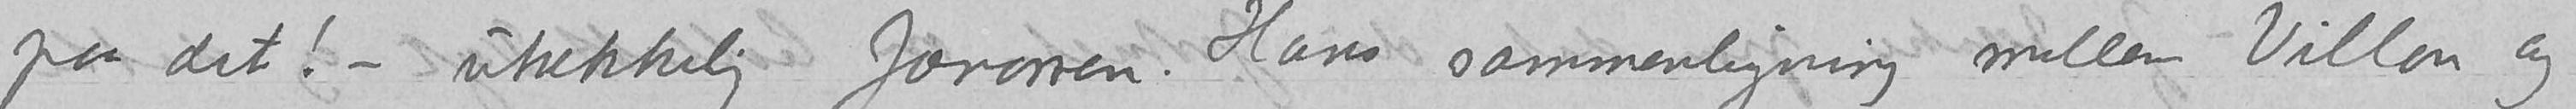paa det! – <utækkelig fænomen. Hans sammenligning mellem Villon og
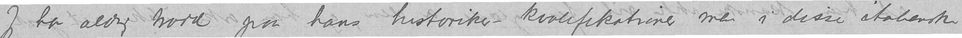Jeg har aldrig trodd paa hans historiker-kvalifikationer men i disse italienske
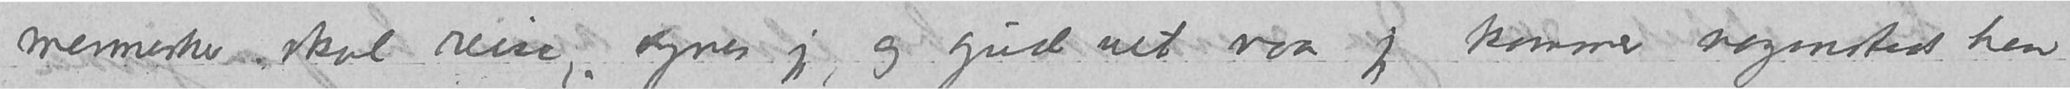mennesker skal reise, synes jeg, og gud vet naar jeg kommer nogensteds hen
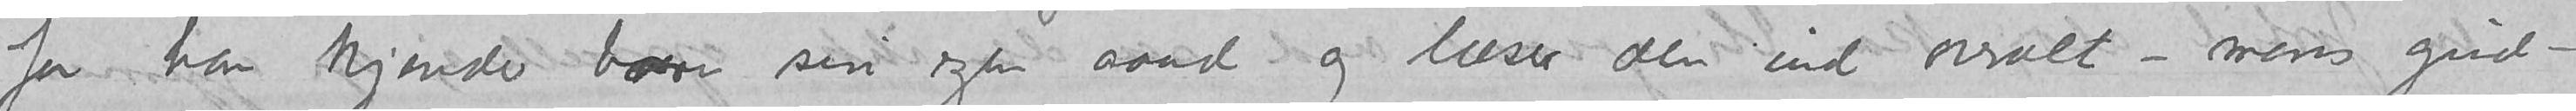for han kjender bare sin egen aand og læser den ind overalt – mens gud-
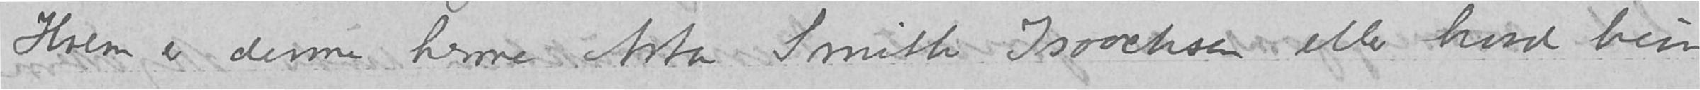Hvem er denne herre Asta Smith Isaachsen eller hvad hun
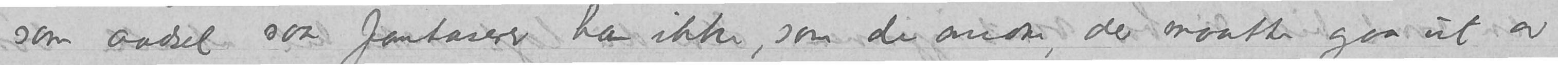som aadsel saa fantaserer han ikke, som de andre, der maatte gaa ut av
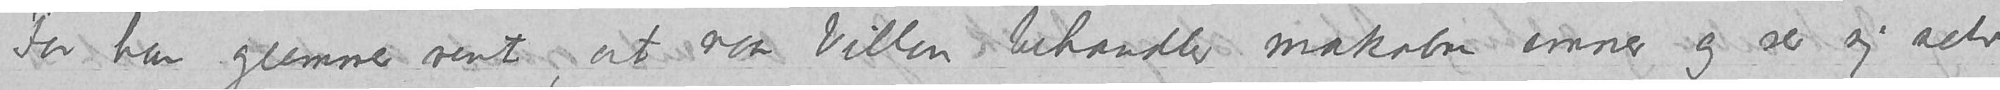For han glemmer rent, at naar Villon behandler makabre emner og ser sig selv
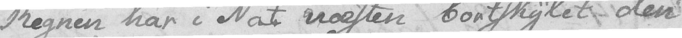Regnen har i Nat næsten bortskylet den
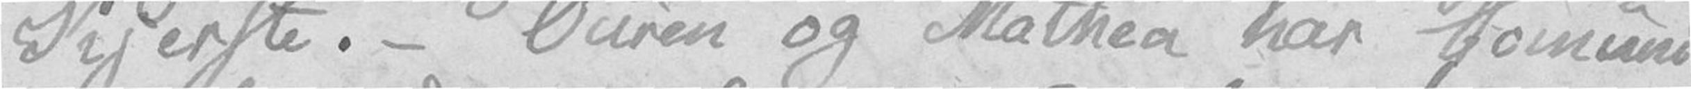Kjerste. – Ouren og Mathea har Comuni
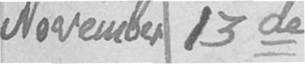November 13de
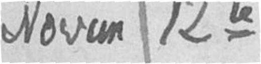Novem 12te
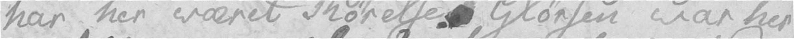har her været Rørelse. Glørsen var her
